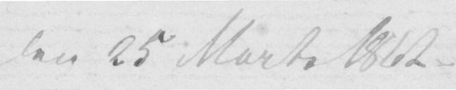Den 25 Marts 1862
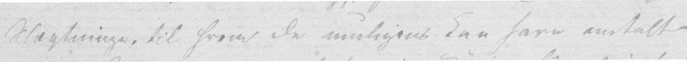Slægtninge, til hvem De muligens kan have omtalt
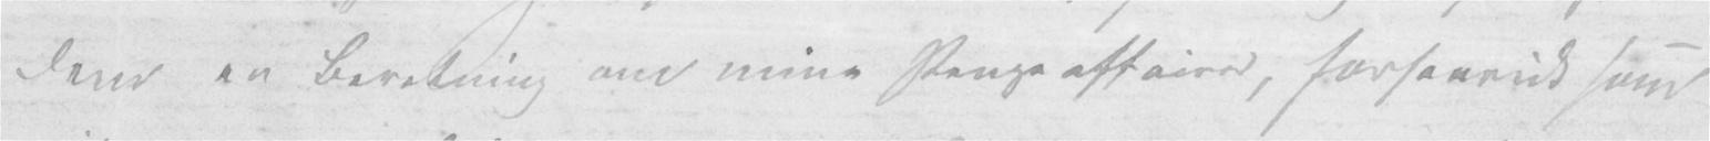Dem en Beretning om mine Pengeaffairer, forsaavidt som
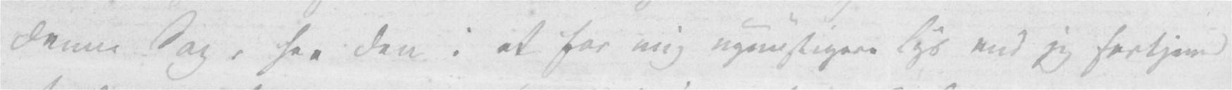denne Sag, see den i et for mig ugunstigere Lys end jeg fortjener
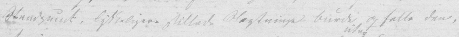Standpunct, lykkeligere stillede Slægtninge burde opfatte den,
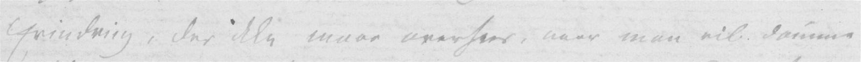Erindring, der ikke maae oversees, naar man vil dømme
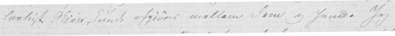lovligt Skjøn, kunde afgjøres mellem Dem og ham. Jeg
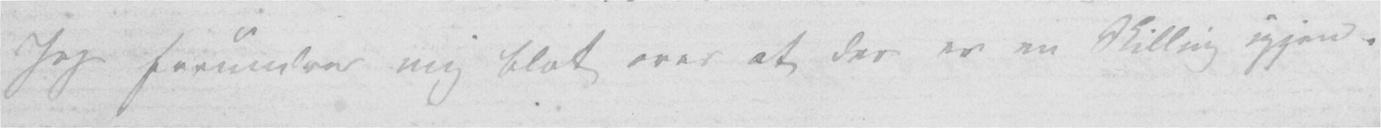Jeg forundrer mig blot over at der er en Skilling igjen.
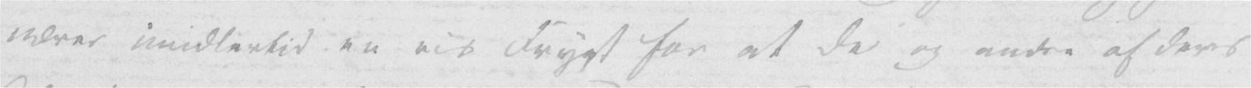nærer imidlertid en vis Frygt for at De og andre af Deres
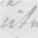utro
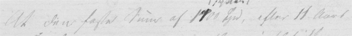At den faste Sum af 1700 Spd, efter 11 Aars
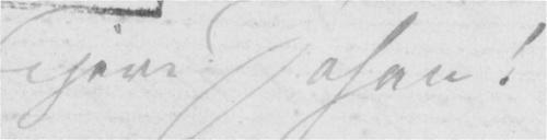Kjere Johan!
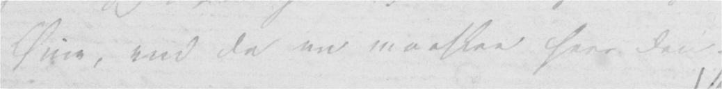Øine, end De nu maaskee seer den.
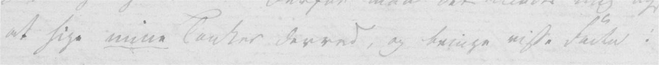at sige mine Tanker derved, og bringe visse Facta i
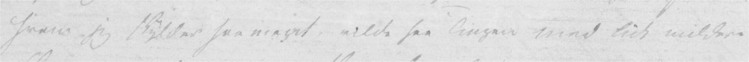hvem jeg skylder saa meget, vilde see Tingen med lidt mildere
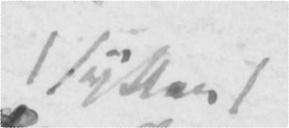(sytten)
